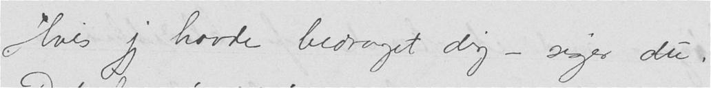Hvis jeg hadde bedraget dig - siger du.
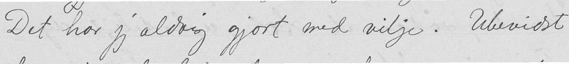Det har jeg aldrig gjort med vilje. Ubevidst
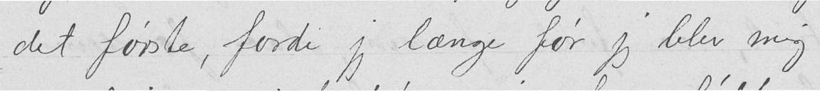det første, fordi jeg længe før jeg blev mig
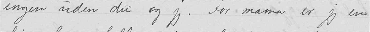ingen uden du og jeg. For mama er jeg en
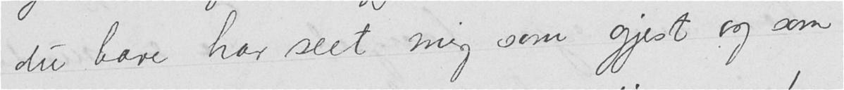du bare har seet mig som gjest og som
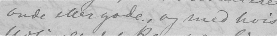onde eller gode, og med hvis
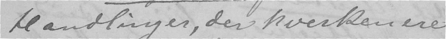Handlinger, der hverken ere
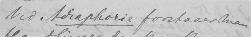Ved Adiaphorie forstaaer man
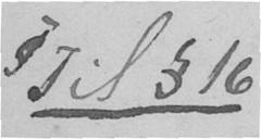Til § 16.
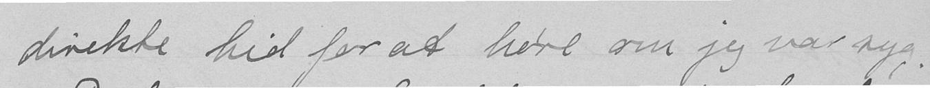direkte hid for at høre om jeg var syg.
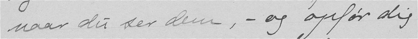naar du ser dem,- og opfør dig
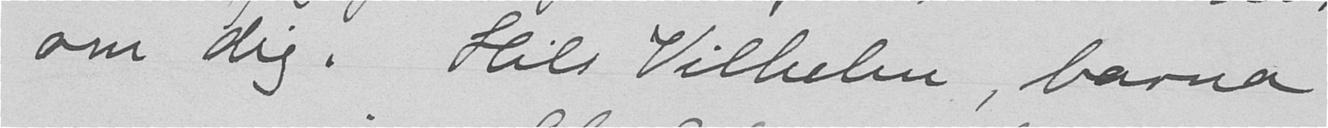om dig. Hils Vilhelm, barna
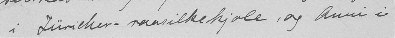i Iuricher-raasilkekjole, og Anni i
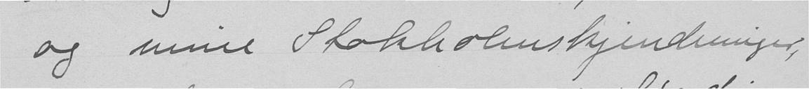og mine Stokholmskjendninger,
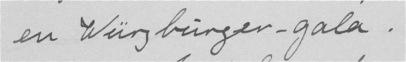en Würzburger -gala.
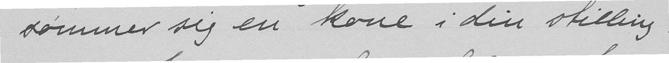sømmer sig en kone i din stilling.
